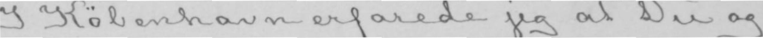I København erfarede jeg at Du og
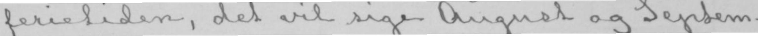ferietiden, det vil sige August og Septem-
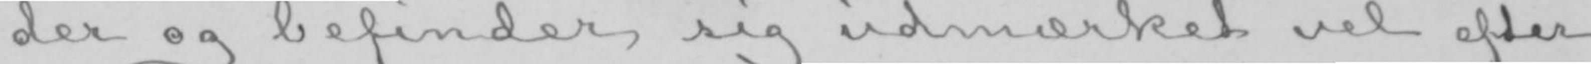der og befinder sig udmærket vel efter
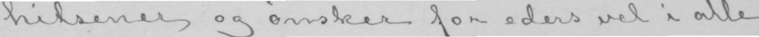hilsener og ønsker for eders vel i alle
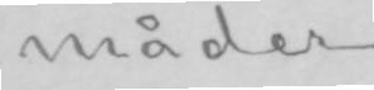måder.
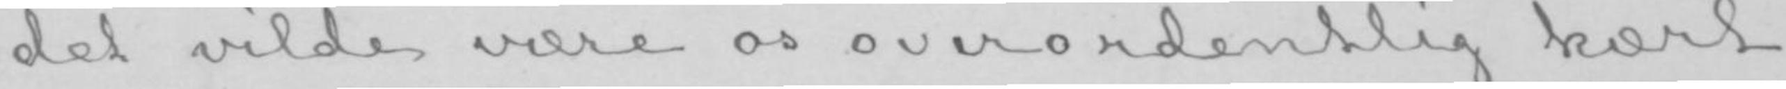det vilde være os overordentlig kært
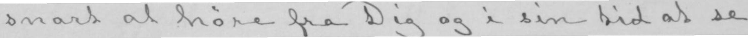snart at høre fra Dig og i sin tid at se
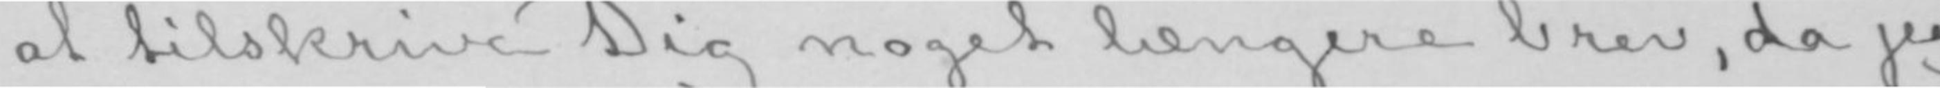at tilskrive Dig noget længere brev, da jeg
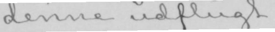denne udflugt.
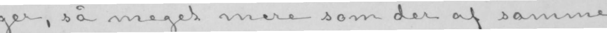ger, så meget mere som der af samme
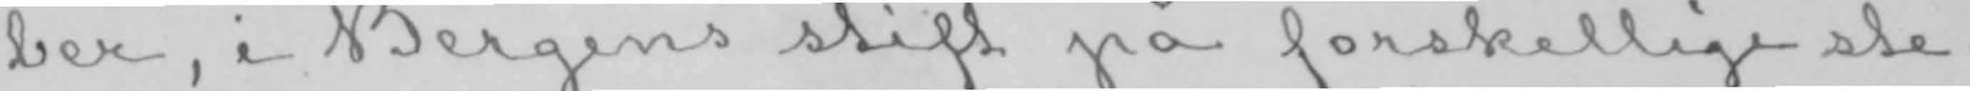ber, i Bergens stift på forskellige ste-
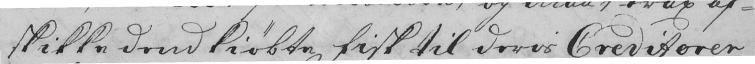skikke dend kiøbte Fisk til deris Creditorer
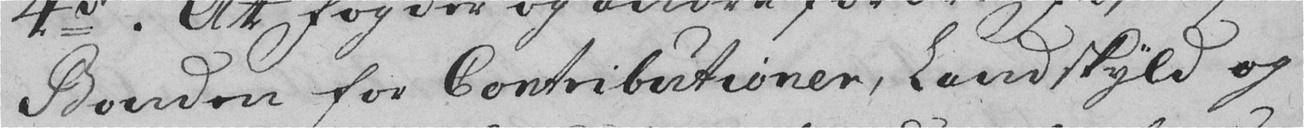Bonden for Contributioner, Landskyld og
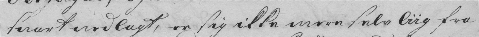snart nedlagt, er sig ikke mere selv liig fra
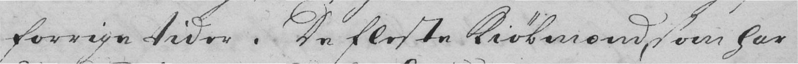forrige tider. De fleste Kiøbmænd, som har
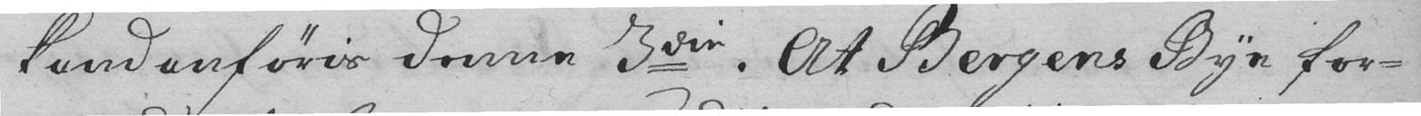kand anføris denne 3die. At Bergens Bye for-
